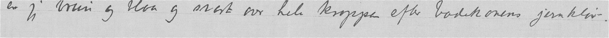er jeg brun og blaa og svart over hele kroppen efter badekonens jernklør.
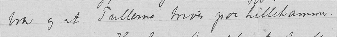bra og at Tullerne trives paa Lillehammer.
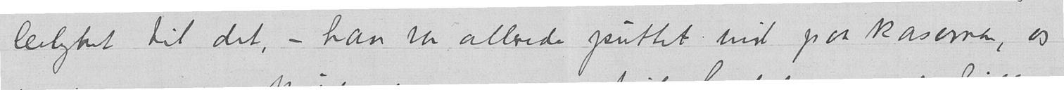leilighet til det, – han var allerede puttet ind paa kasernen, og
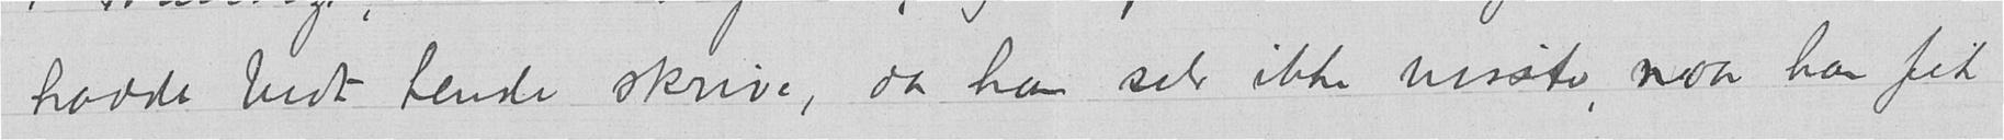hadde bedt hende skrive, da han selv ikke visste, naar han fik,
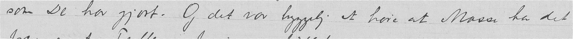som De har gjort. Og det var hyggelig at høre at Mosse har det
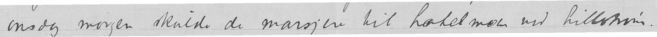onsdag morgen skulde de marsjere til Lahelmoen ved Lillestrøm.
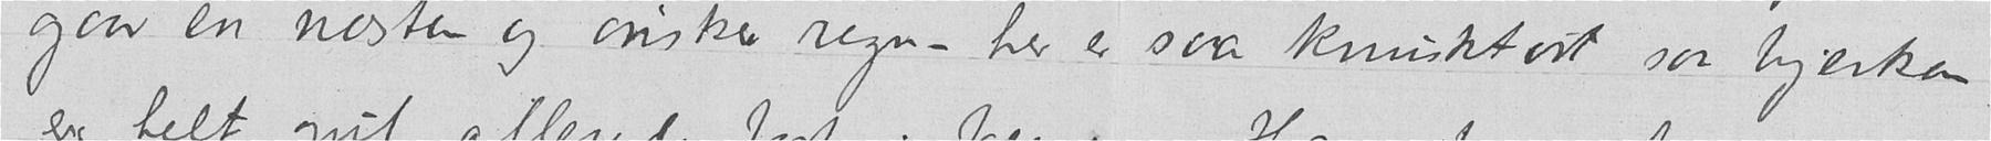gaar en næsten og ønsker regn – her er saa knusktørt saa bjerken
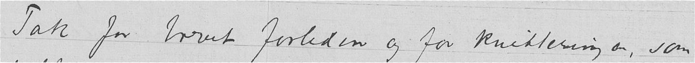Tak for brevet forleden og for kvitteringen, som
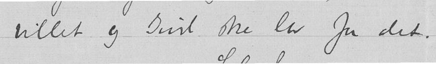villet og Gud ske lov for det.
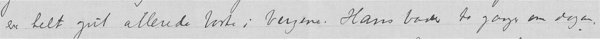er helt gul allerede borte i bergene. Hans bader to ganger om dagen,
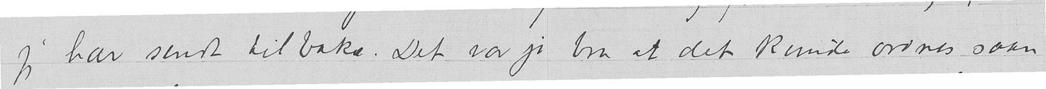jeg har sendt tilbake. Det var jo bra at det kunde ordnes saan
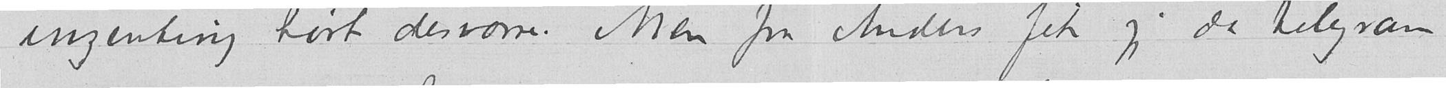ingenting hørt desværre. Men fra Anders fik jeg da telegram
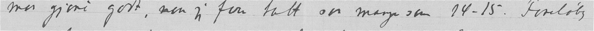maa gjøre godt, naar jeg faar tatt saa mange som 14-15. Foreløbig
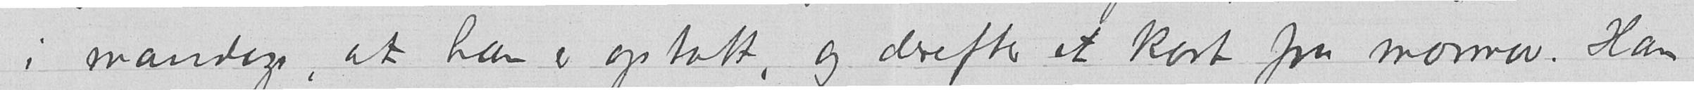i mandags, at han er optatt, og derefter et kort fra mormor. Han
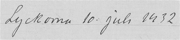Lyckoma 10. juli 1932
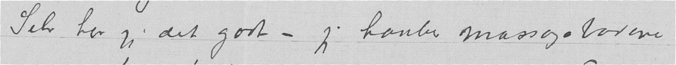Selv har jeg det godt – jeg haaber massagebadene
